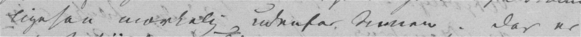ligesaa mærkelig udenfor Scenen. Der er
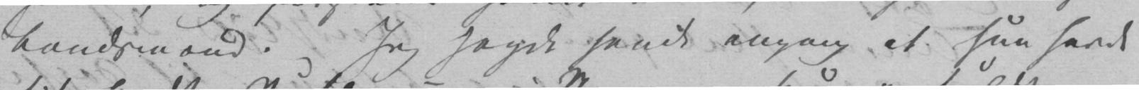Landsmænd. Jeg sagde hende engang at hun havde
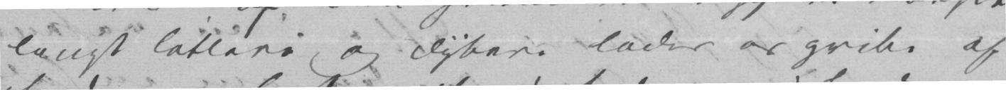langt lettere og dybere lader os gribe af
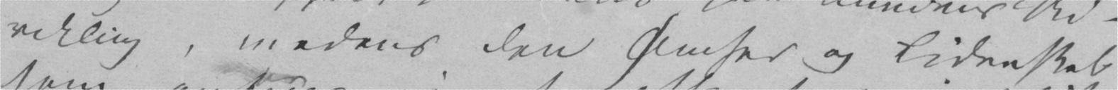vikling, medens den Ømhed og Lidenskab
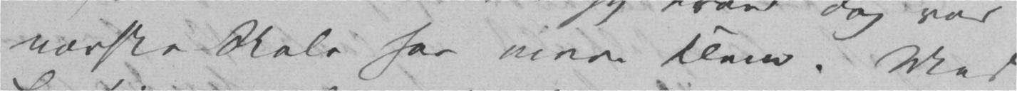norske Skole har mere Klem. Med
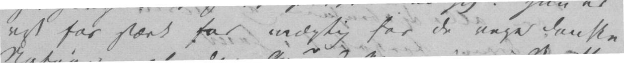vist for stærk for mægtig for de unge danske
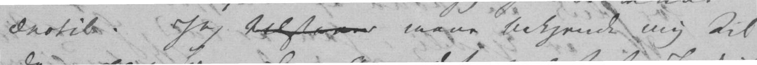dertil. Jeg tilstaaer maae bekjende mig til
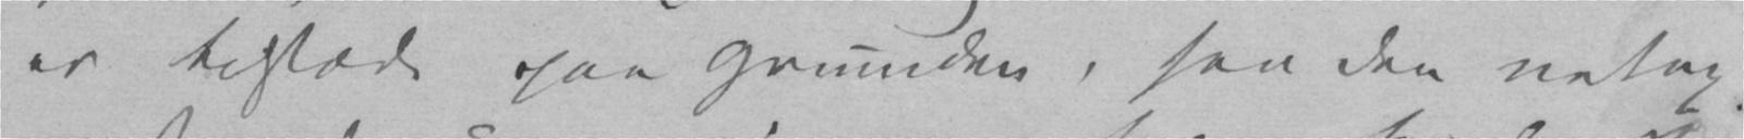er tilstæde paa Grunden, saa den netop
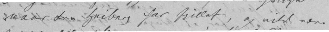naar Fru Heiberg har spillet, og vilde være
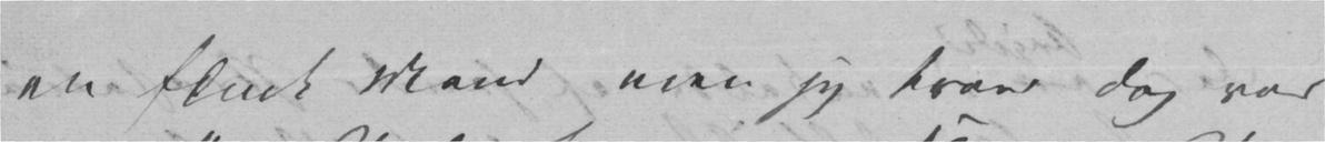en flink Mand men jeg troer dog vor
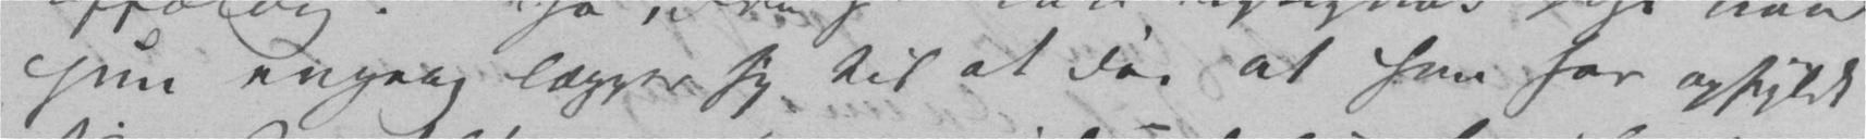hun engang lægger sig til at døe at hun har opfyldt
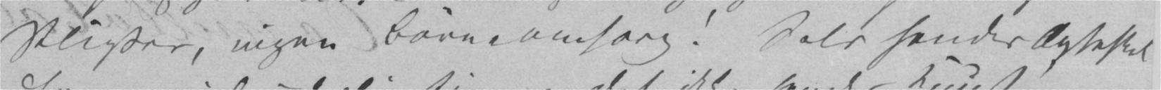Pligter, ingen Børneomsorg! Selv hendes Ægteskab
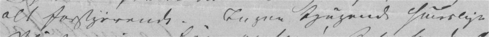alt forstyrrende. Ingen tyngende huuslige
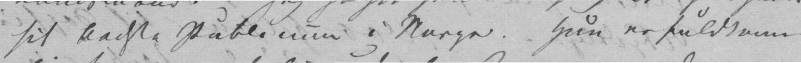sit bedste Publicum i Norge. Hun er fuldkommen
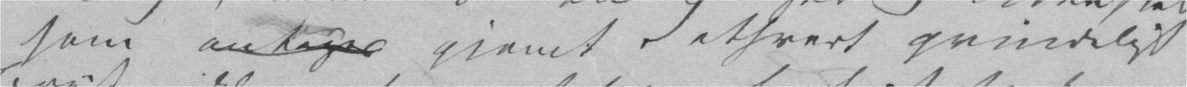som antages er gjemt i ethvert qvindeligt
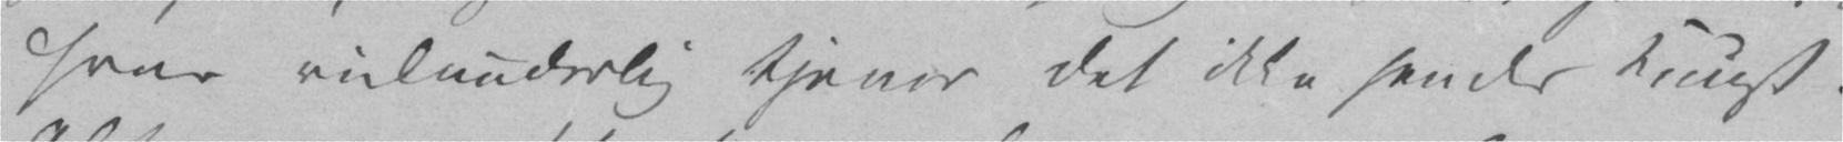hvor vidunderlig tjener det ikke hendes Kunst!
